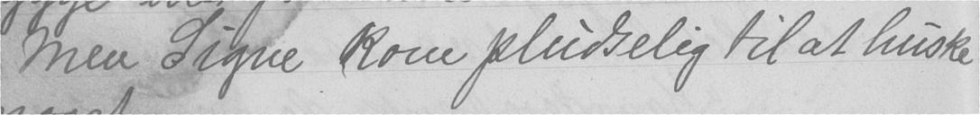men Signe kom pludselig til at huske
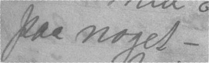paa noget -
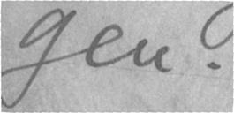gen.
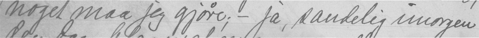noget maa jeg gjøre; - ja, sandelig imorgen
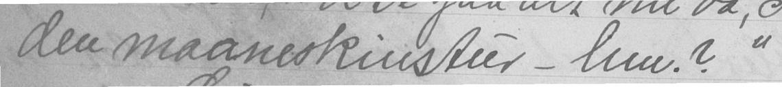den maaneskinstur - hm.?"
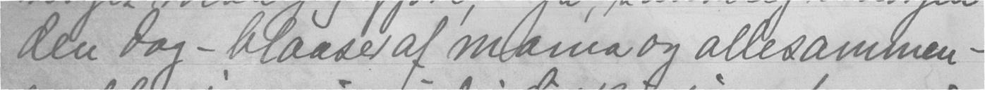den dag - blaaser af mama og allesammen -
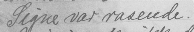Signe var rasende.
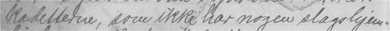kadetterne, som ikke har nogen slags hjem-
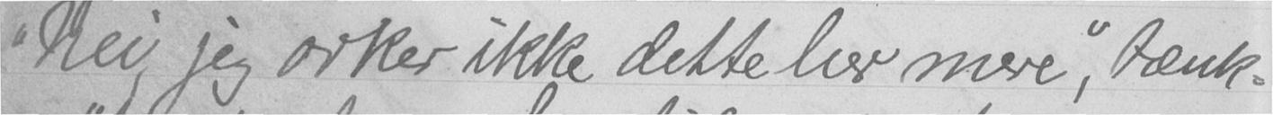"Nei, jeg orker ikke dette her mere," tænk-
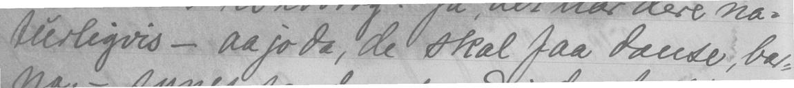turligvis - aa jo da, de skal faa danse, bar-
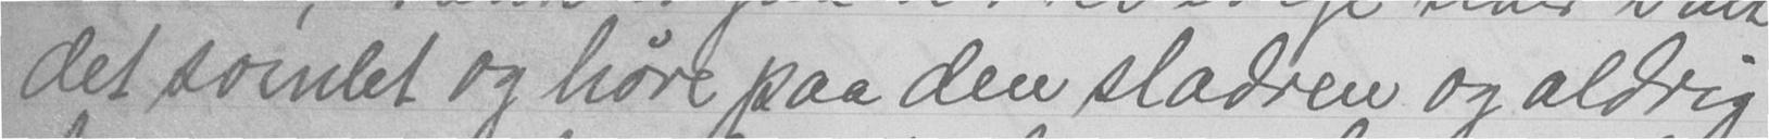det somlet og høre paa den sladren og aldrig
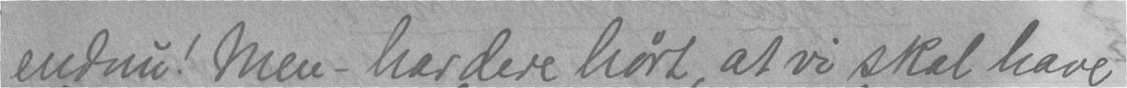endnu! Men - har dere hørt, at vi skal have
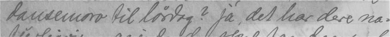dansemoro til lørdag? ja, det har dere na-
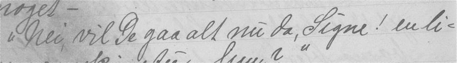"Nei, vil De gaa alt nu da, Signe! en li-
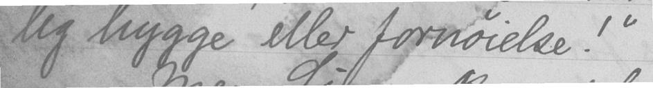lig hygge eller fornøielse!"
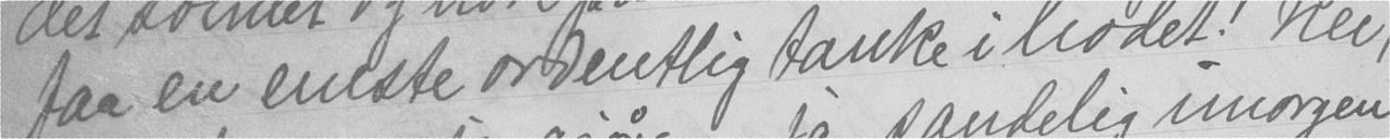faa en eneste ordentlig tanke i hodet! Nei,
